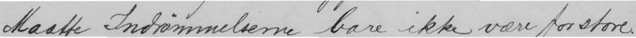Maatte Indrømmelserne bare ikke være for store !
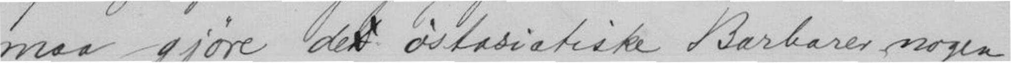maa gjøre de østasiatiske Barbarer nogen
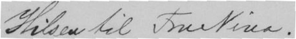Hilsen til Fru Nina.
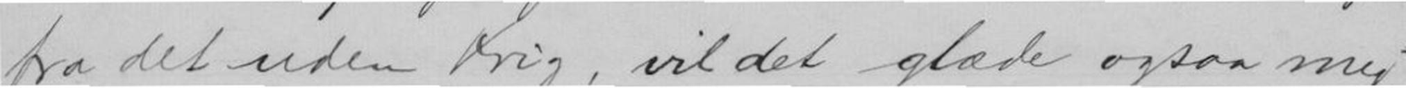fra det uden Krig, vil det glæde ogsaa mig
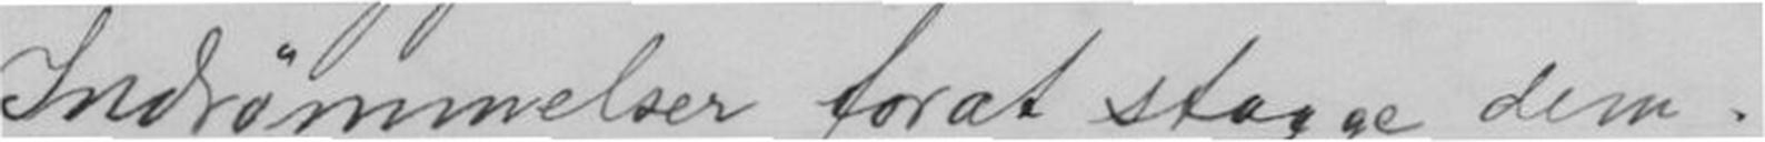Indrømmelser forat stagge dem.
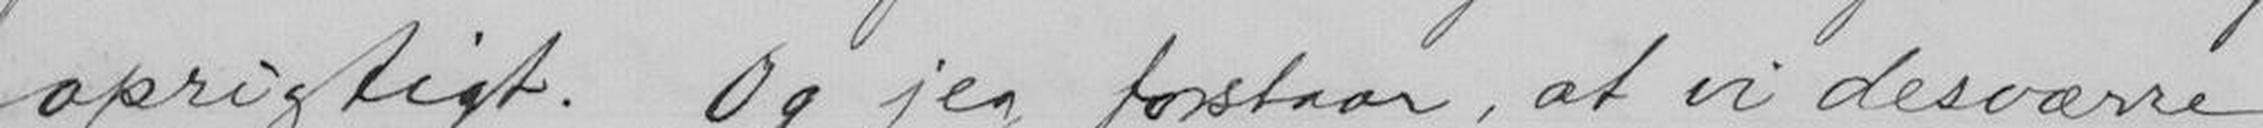oprigtigt. Og jeg forstaar, at vi desværre
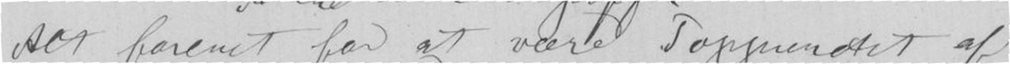Alt forenet for at være Topprunctet af
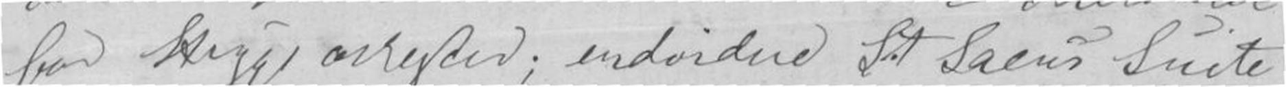for Strygeorkester; endvidere Snt Saëns Suite
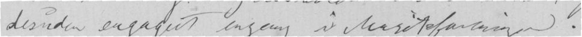desuden engagert engang i Musikforeningen.
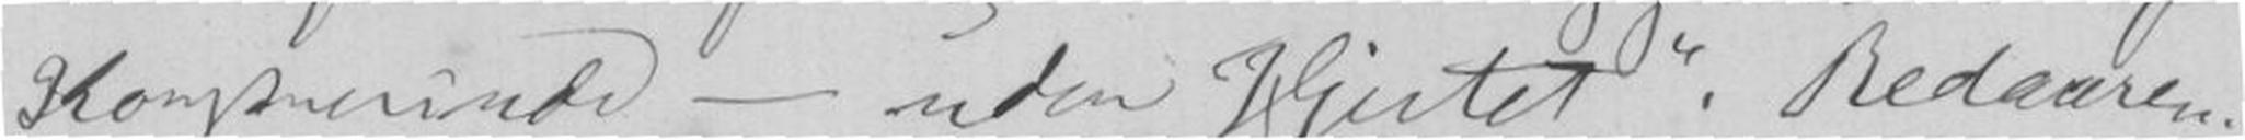Konstnerinde - uden Hjertet. Bedaaren-
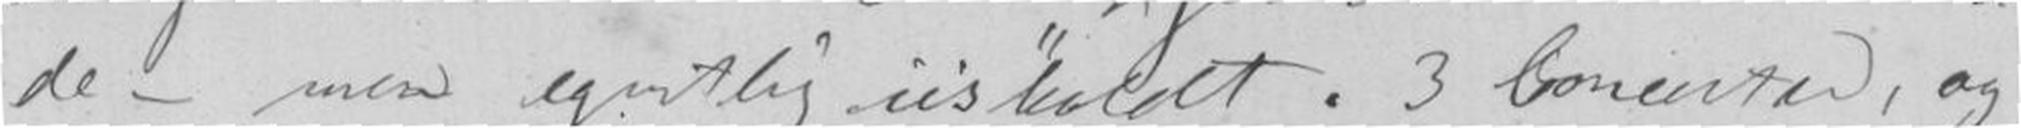de - men egentlig iskaldt. 3 Concerter, og
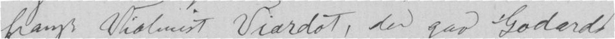fransk Violinist Viardot, der gav Godards
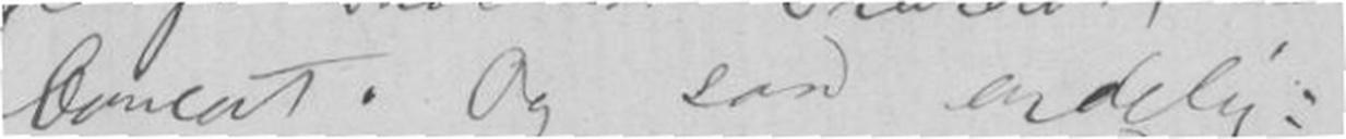Concert. Og saa endelig:
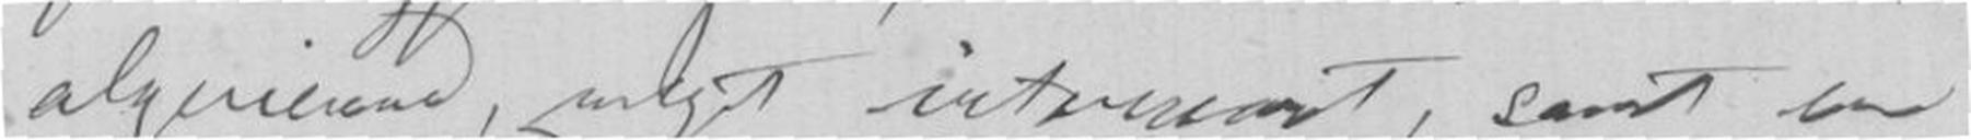algerienne, meget intressant, somt en
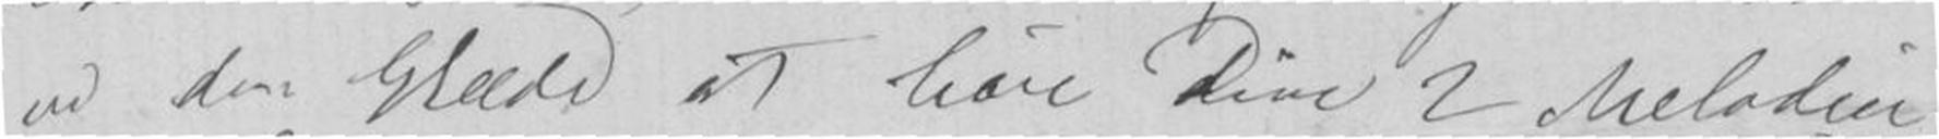vi den Glæde at høre Dine 2 Melodier
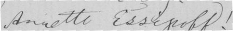Annette Essepoff!
